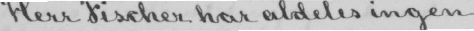Herr Fischer har aldeles ingen
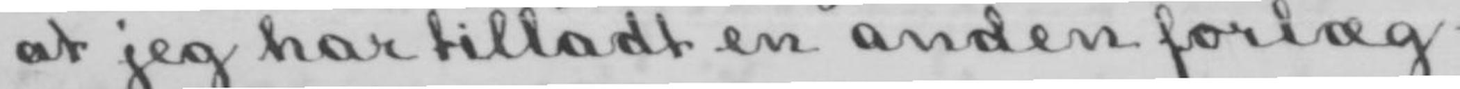at jeg har tilladt en anden forlæg-
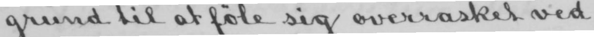grund til at føle sig overrasket ved
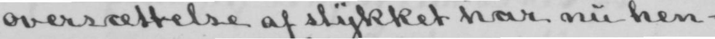oversættelse af stykket har nu hen-
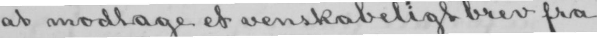at modtage et venskabeligt brev fra
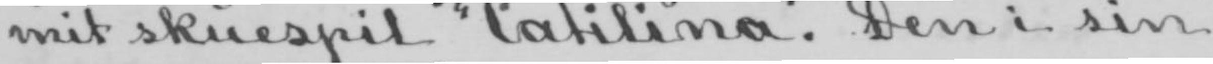mit skuespil «Catilina». Den i sin
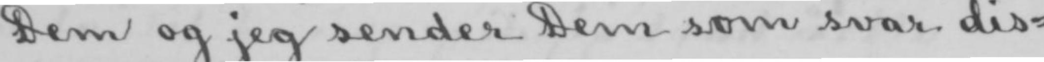Dem og jeg sender Dem som svar dis-
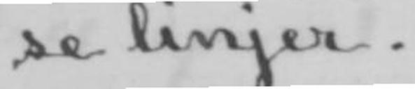se linjer.
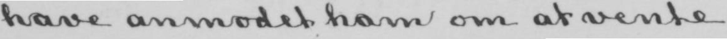have anmodet ham om at vente
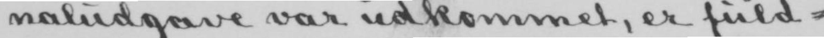naludgave var udkommet, er fuld-
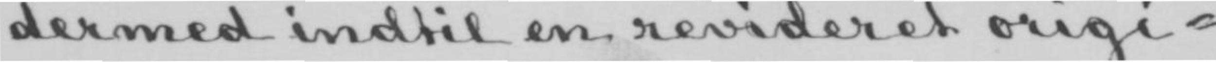dermed indtil en revideret origi-
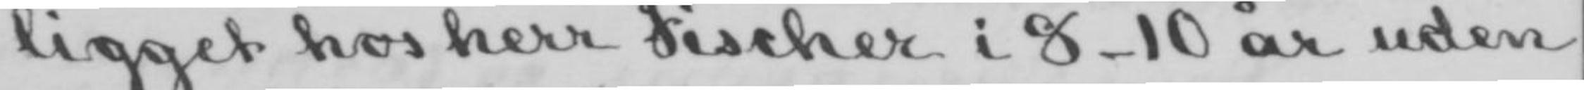ligget hos herr Fischer i 8-10 år uden
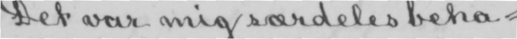Det var mig særdeles beha-
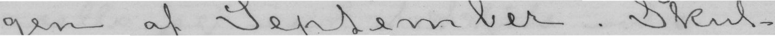gen af September. Skul-
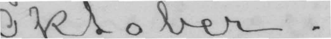Oktober.
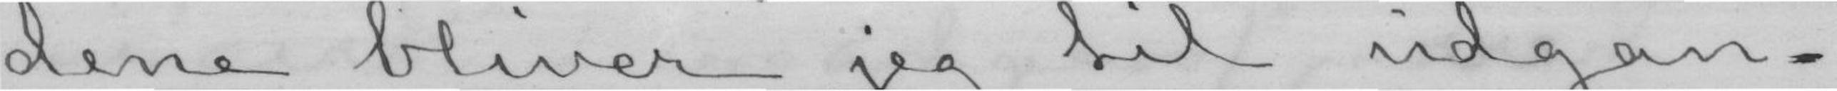dene bliver jeg til udgan-
